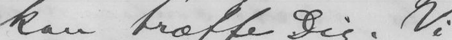kan træffe Dig. Vi
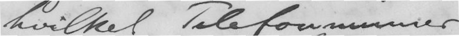hvilket Telefonnummer,
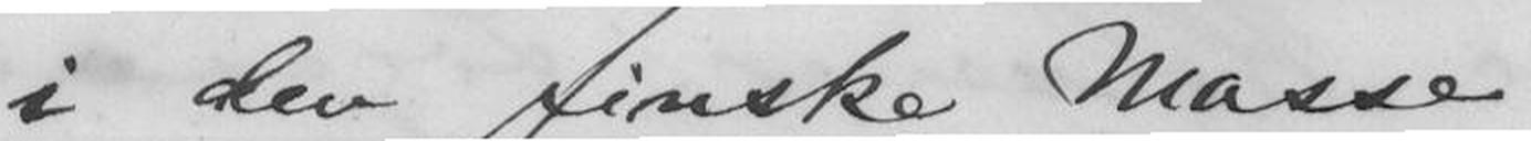i den finske Masse.
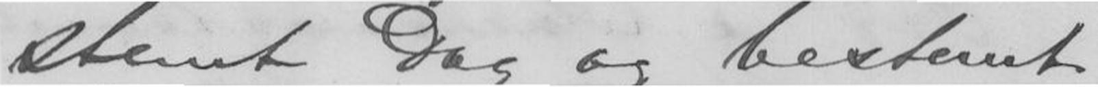stemt Dag og bestemt
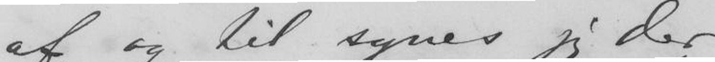af og til synes jeg der
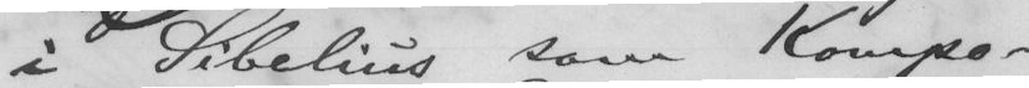i Sibelius som Kompo-
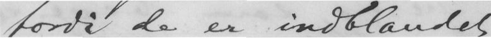fordi de er indblandet
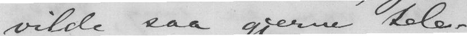vilde saa gjerne tele-
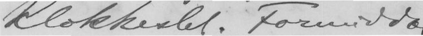Klokkeslet. Formiddagen
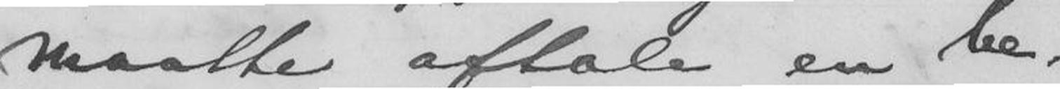maatte aftale en be-
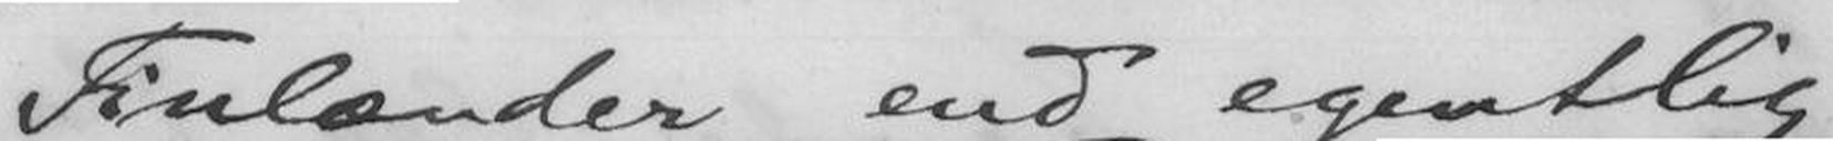Finlænder end egentlig
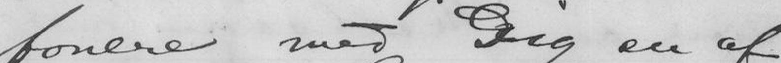fonere med Dig en af
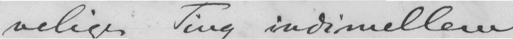velige Ting indimellem,
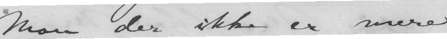Mon der ikke er mere
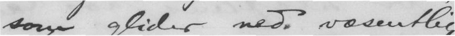som glider ned væsentlig
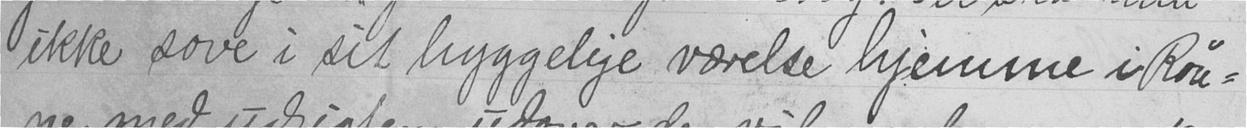ikke sove i sit hyggelige værelse hjemme i Røn-
